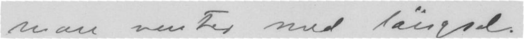man venter med längsel.
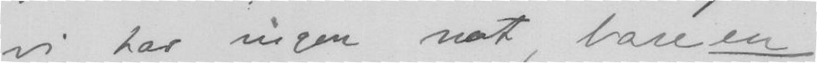vi har ingen nat, bare en
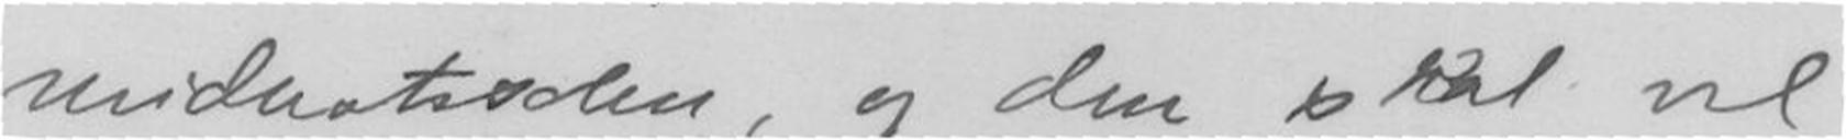midnatsolen, og den skal vel
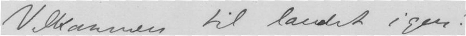Velkommen til landet igjen!
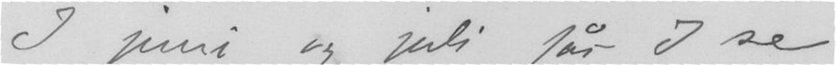I juni og juli får I se
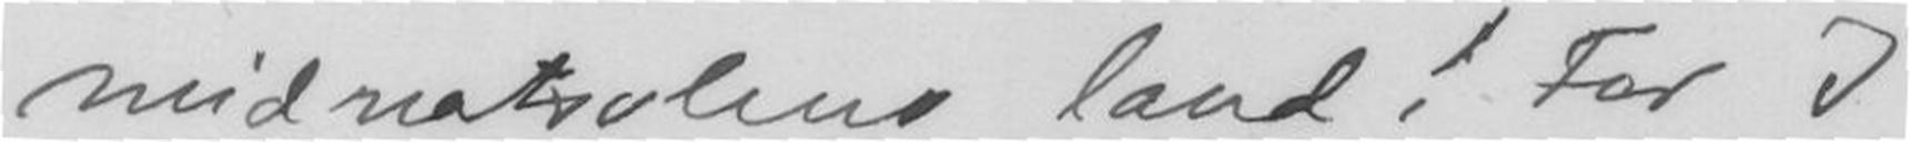midnatsolens land! For I
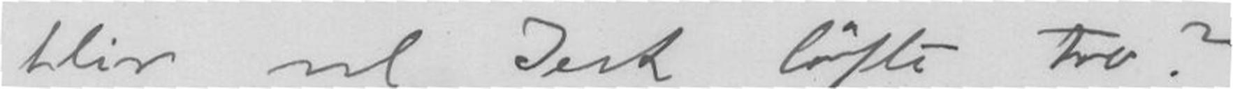blir vel Jert løfte tro?
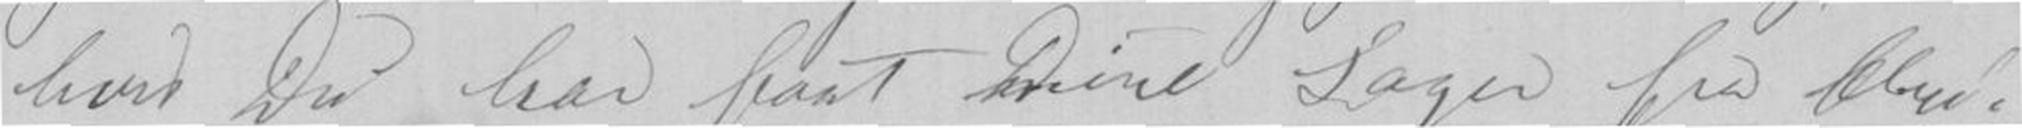hvis Du har faat Dine Sager fra Chri-
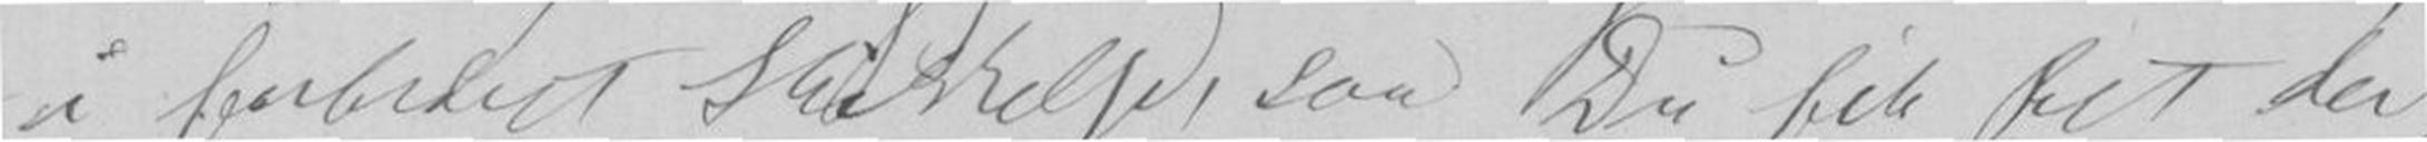i forbedret Skikkelse, saa Du fik Ret der,
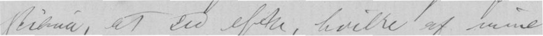stiania, at see efter, hvilke af mine
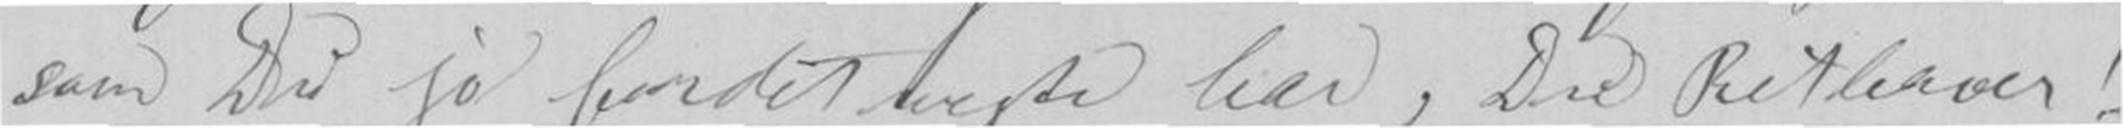som Du jo for det meste har, Du Rethaver!
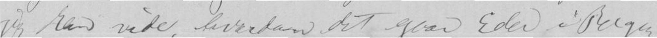jeg kan vid, hvordan det gaar Eder i Bergen,
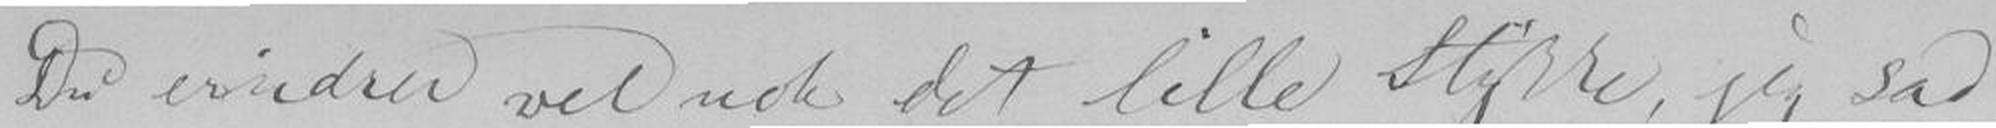Du erinderer vel nok det lille Stykke, jeg sad
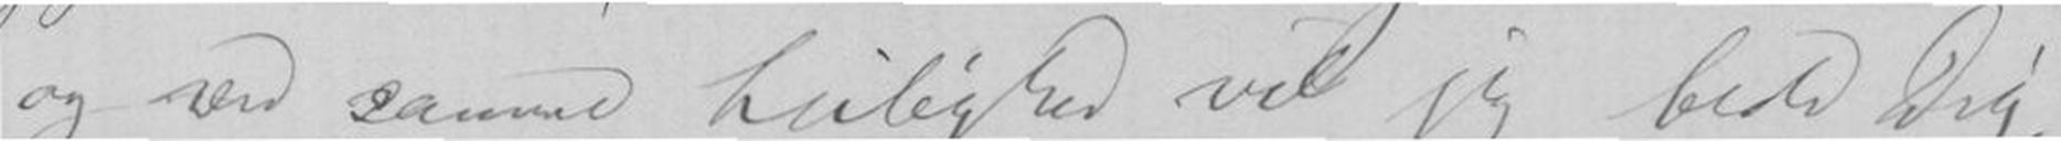og ved samme Leilighed vil jeg bede Dig,
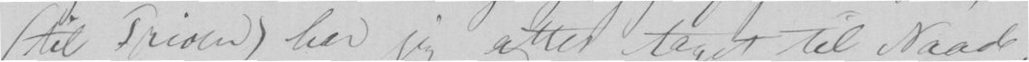(til Trioen) har jeg atter taget til Naade,
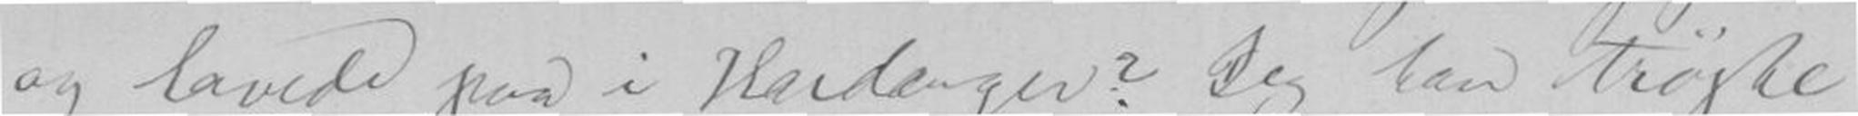og lavede paa i Hardanger? Jeg kan trøste
